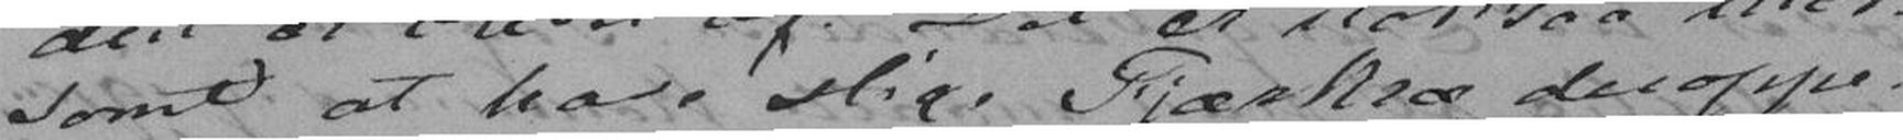somt at have slige Fjærkræ deroppe.
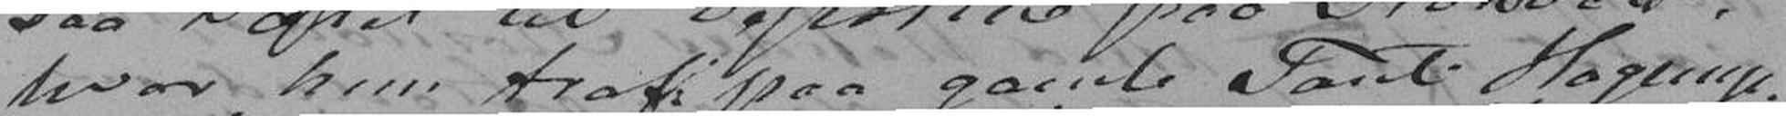hvor hun traf paa gamle Tante Hagerup,
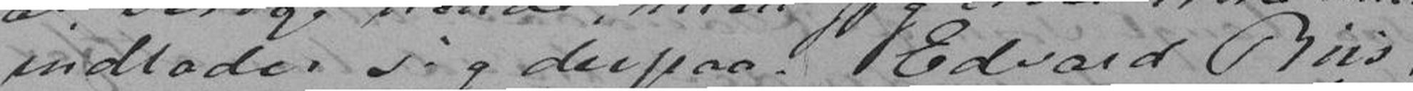indlader sig derpaa. Edvard Riis,
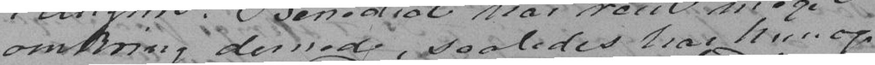omkring dernede, saaledes har hun og-
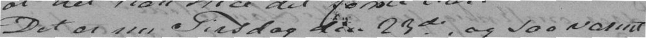Det er nu Tirsdag den 23de, og saa varmt
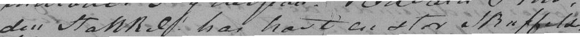den Stakkels har havt en stor skuffelse.
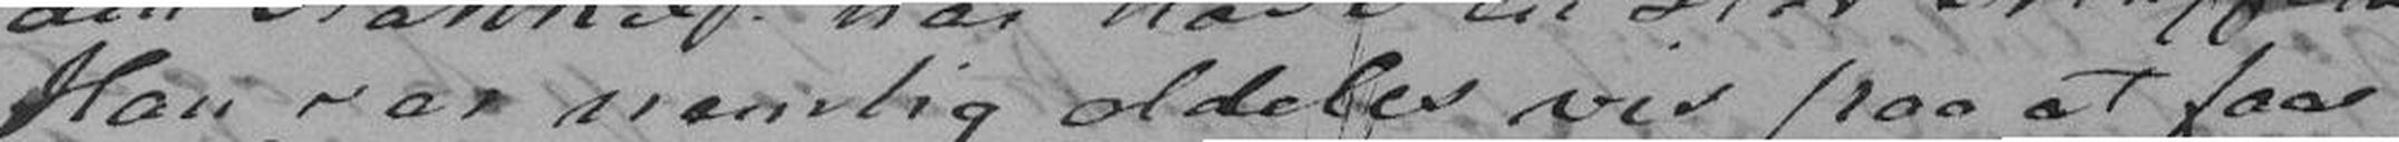Han var nemlig aldeles vis paa at faae
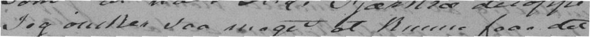Jeg ønsker saa meget at kunne faae det
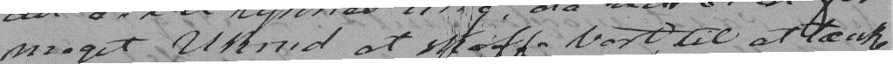meget Ukrud at skaffe bort til at tænke
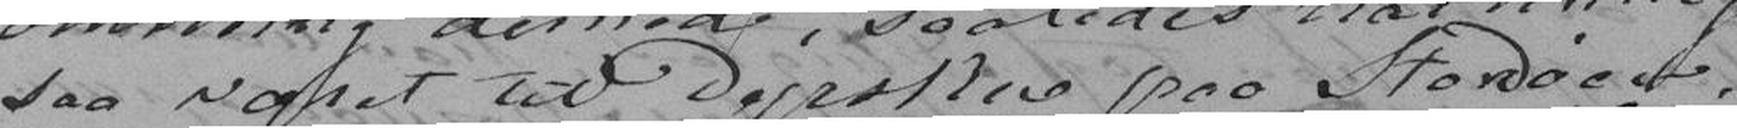saa været til Dyrskue paa Stordøen,
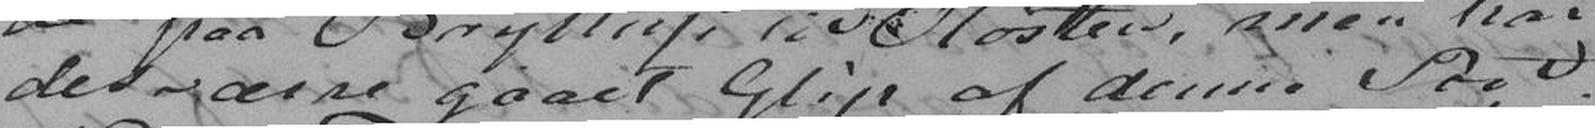desværre gaaet Glip af denne Post.
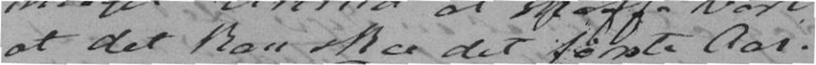at det kan skee det første Aar.
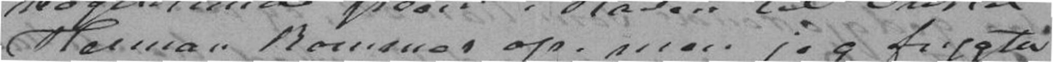Herman kommer op, men jeg frygter
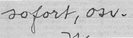sofort, osv.
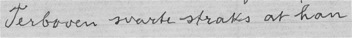Terboven svarte straks at han
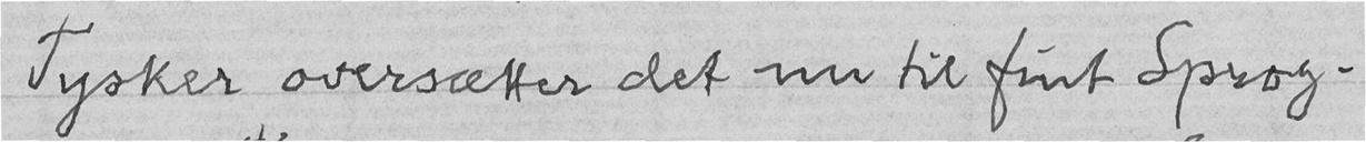Tysker oversætter det nu til fint Sprog.
